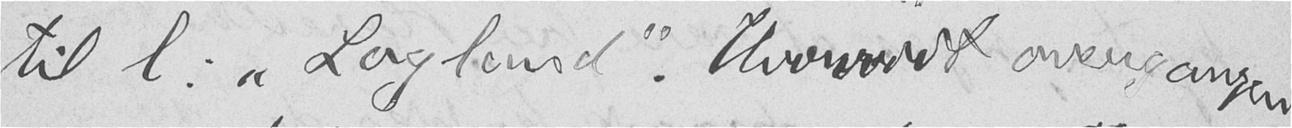til l: "Logland". Hvorvidt overgangen
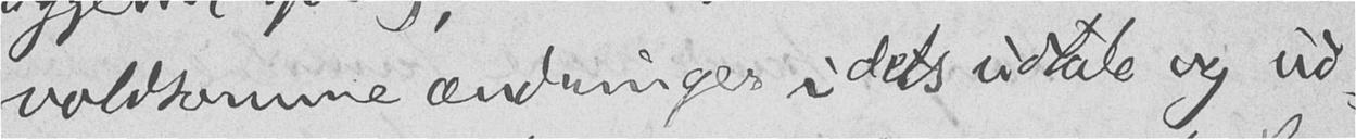voldsomme ændringer i dets udtale og ud-
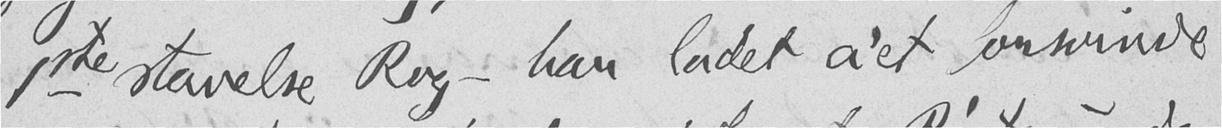1ste stavelse Rog- har ladet a'et forsvinde
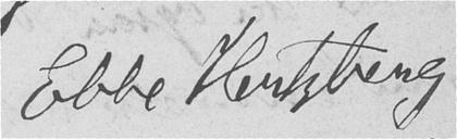Ebbe Hertzberg.
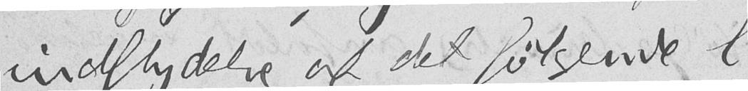indflydelse af det følgende l
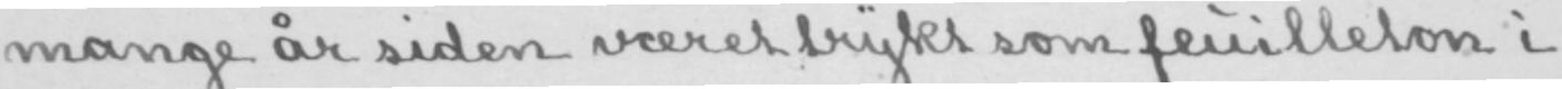mange år siden været trykt som feuilleton i
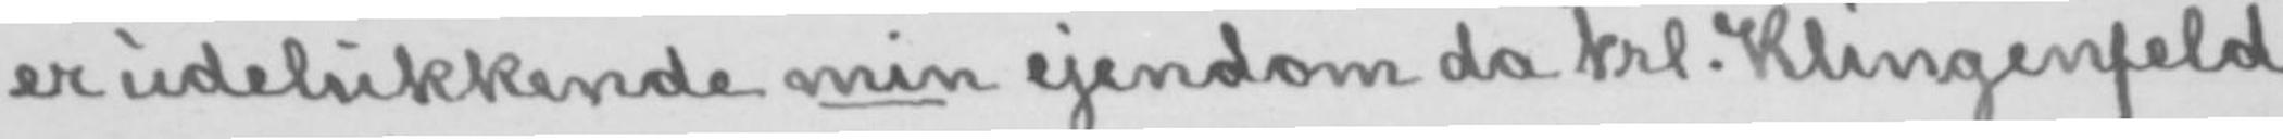er udelukkende min ejendom da Frl. Klingenfeld
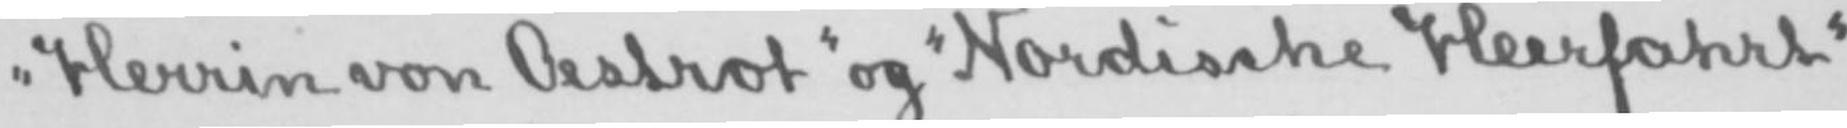«Herrin von Oestrot» og «Nordische Heerfahrt»
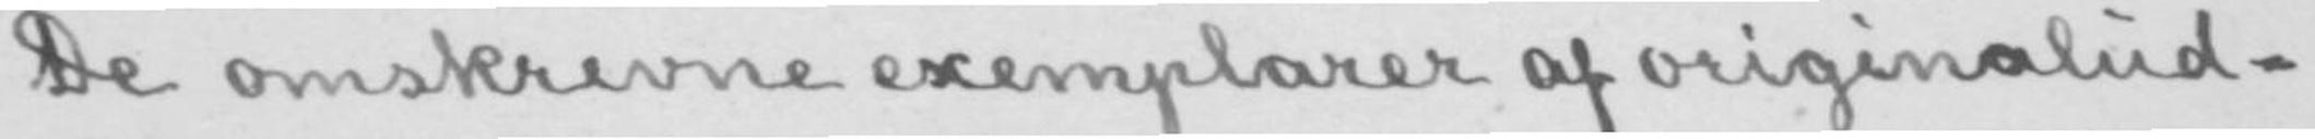De omskrevne exemplarer af originalud-
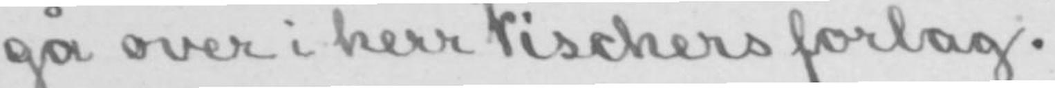gå over i herr Fischers forlag.
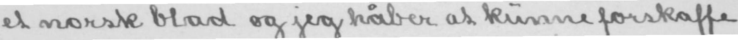et norsk blad og jeg håber at kunne forskaffe
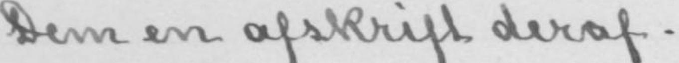Dem en afskrift deraf.
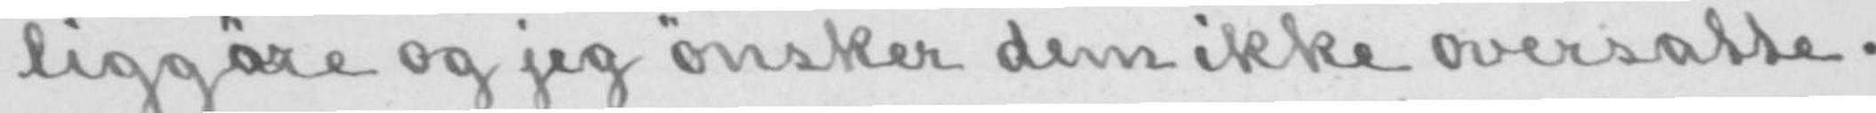liggøre og jeg ønsker dem ikke oversatte.
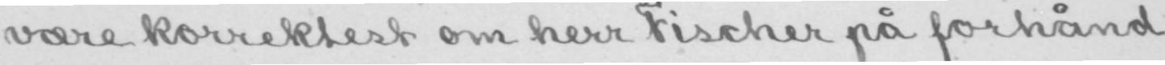være korrektest om herr Fischer på forhånd
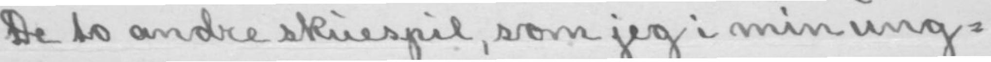De to andre skuespil, som jeg i min ung-
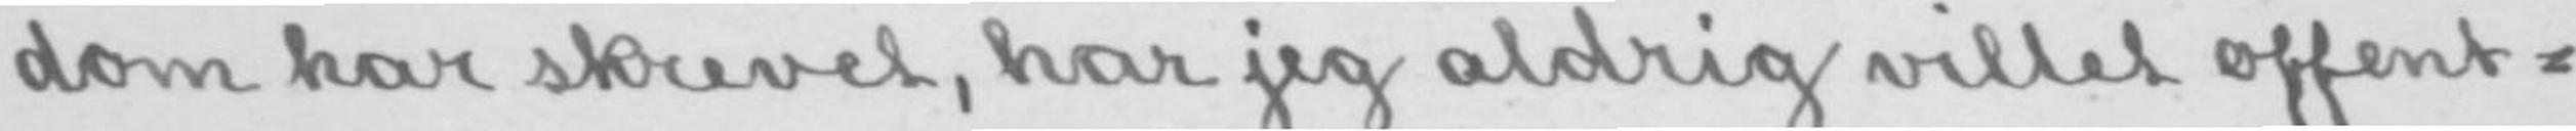dom har skrevet, har jeg aldrig villet offent-
